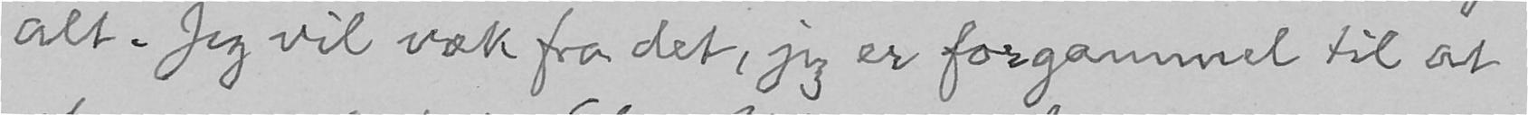alt. Jeg vil væk fra det, jeg er for gammel til at
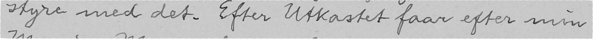styre med det. Efter Utkastet faar efter min
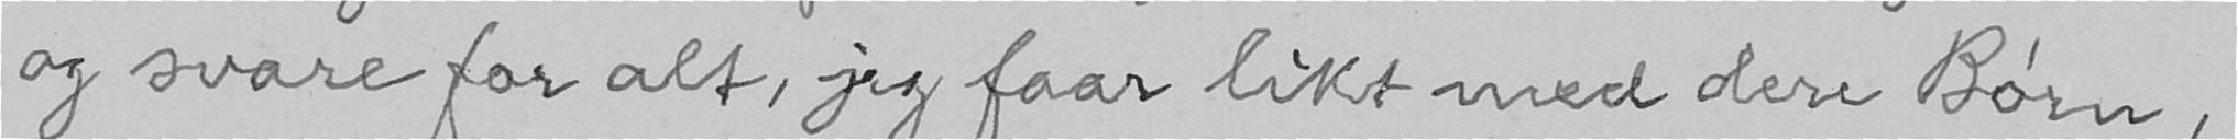og svare for alt, jeg faar likt med dere Børn,
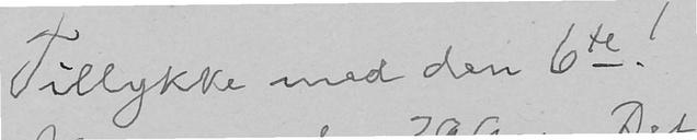Tillykke med den 6te!
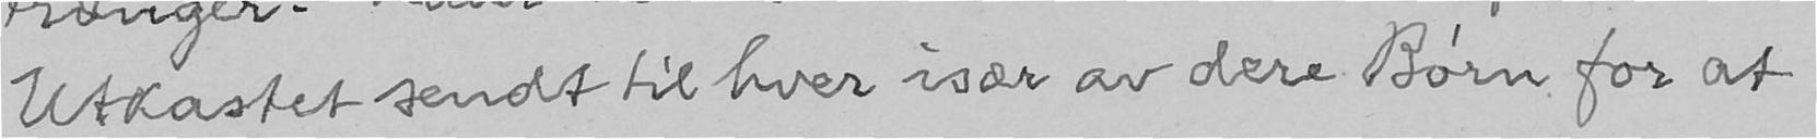Utkastet sendt til hver især av dere Børn for at
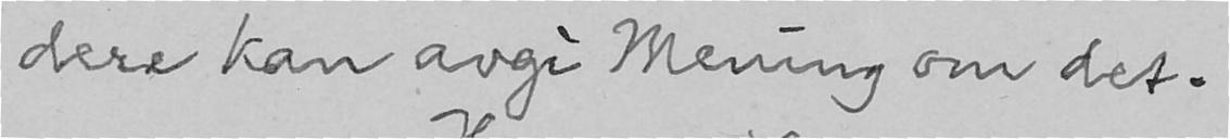dere kan avgi Mening om det.
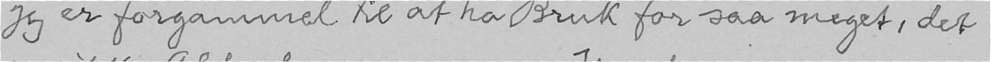jeg er forgammel til at ha Bruk for saa meget, det
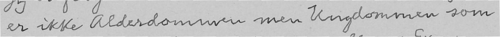er ikke Alderdommen men Ungdommen som
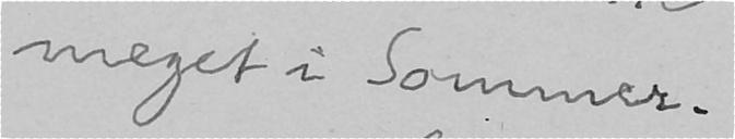meget i Sommer.
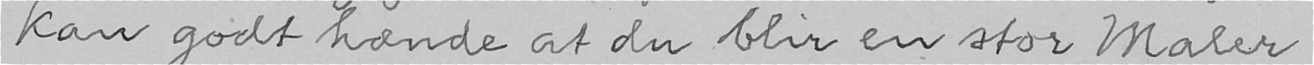kan godt hænde at du blir en stor Maler
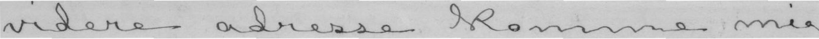videre adresse komme mig
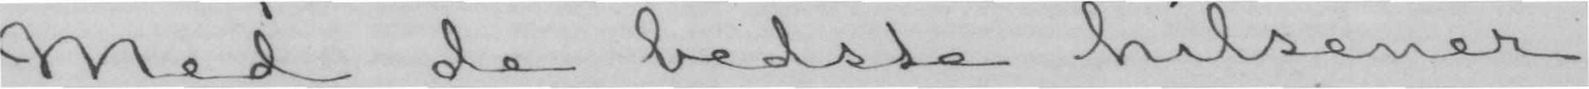Med de bedste hilsener
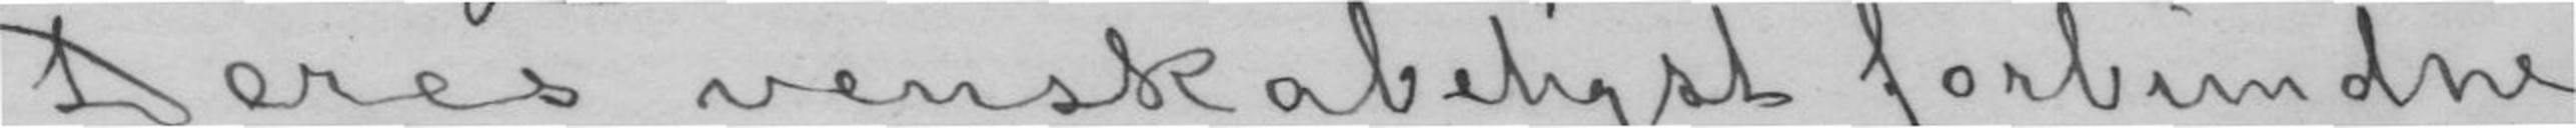Deres venskabeligst forbundne
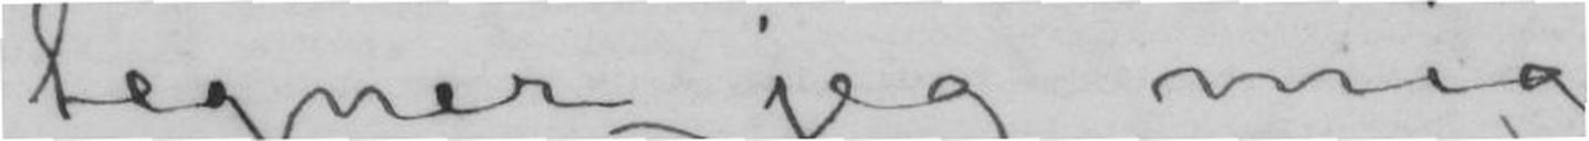tegner jeg mig
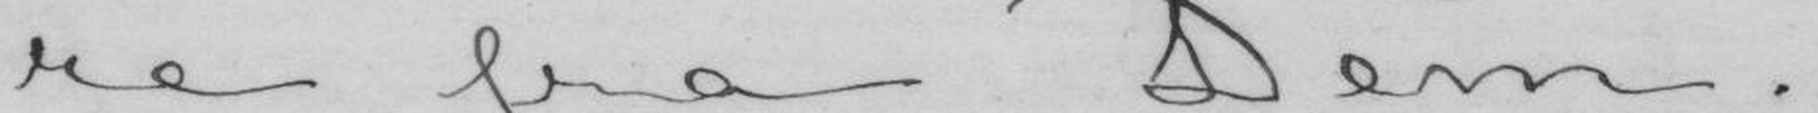re fra Dem.
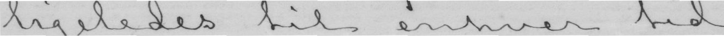ligeledes til enhver tid
